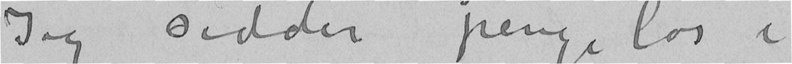Jeg sidder pengeløs i
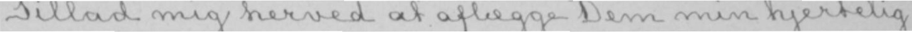Tillad mig herved at aflægge Dem min hjertelig-
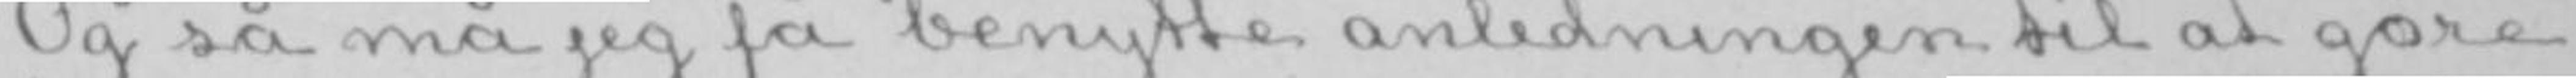Og så må jeg få benytte anledningen til at gøre
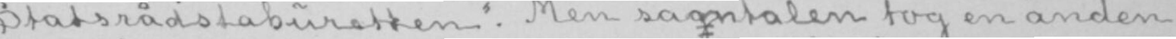Statsrådstaburetten». Men sagmtalen tog en anden
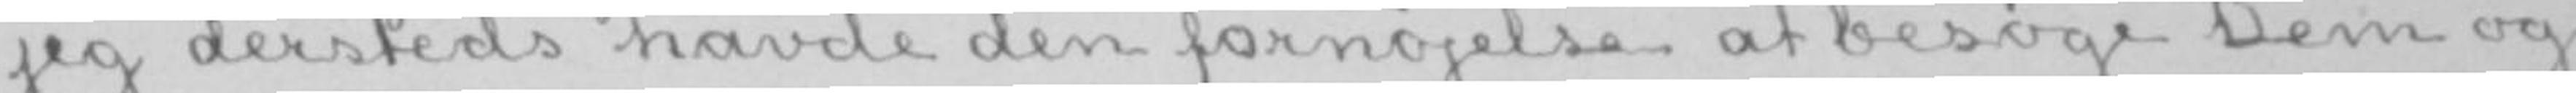jeg dersteds havde den fornøjelse at besøge Dem og
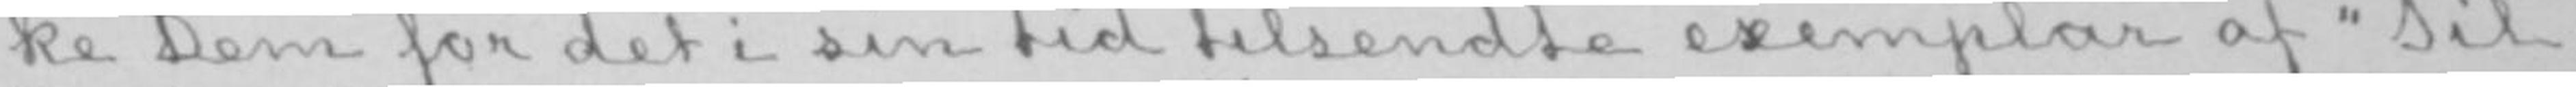ke Dem for det i sin tid tilsendte exemplar af «Til
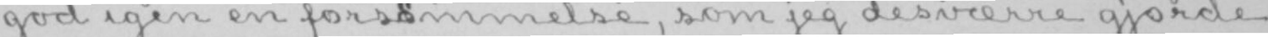god igen en forsømmelse, som jeg desværre gjorde
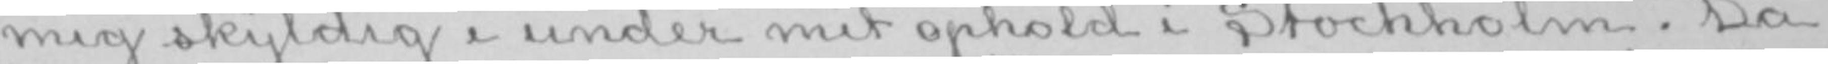mig skyldig i under mit ophold i Stochholm. Da
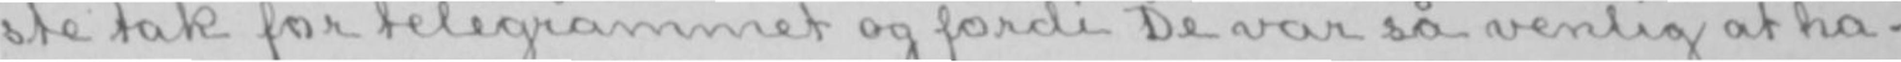ste tak for telegrammet og fordi De var så venlig at ha-
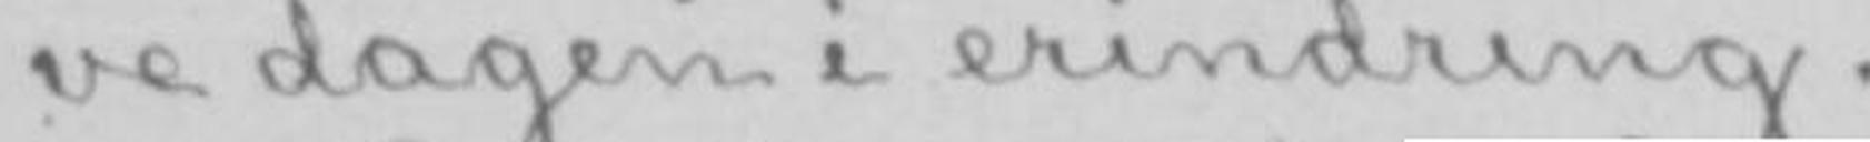ve dagen i erindring.
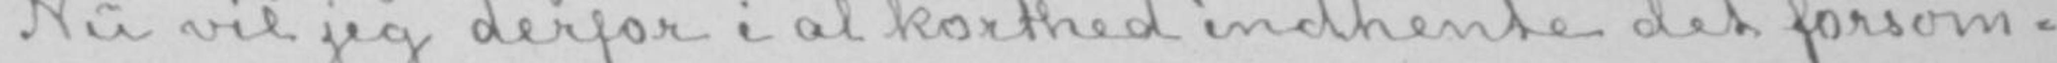Nu vil jeg derfor i al korthed indhente det forsøm-
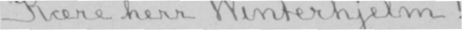Kære herr Winterhjelm!
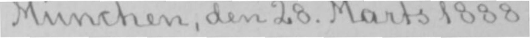München, den 28. Marts 1888.
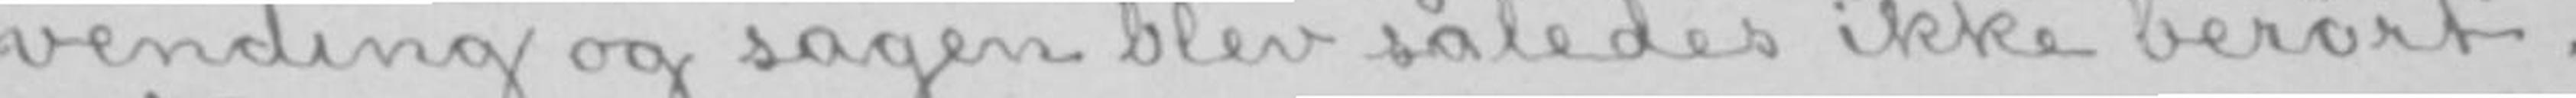vending og sagen blev således ikke berørt.
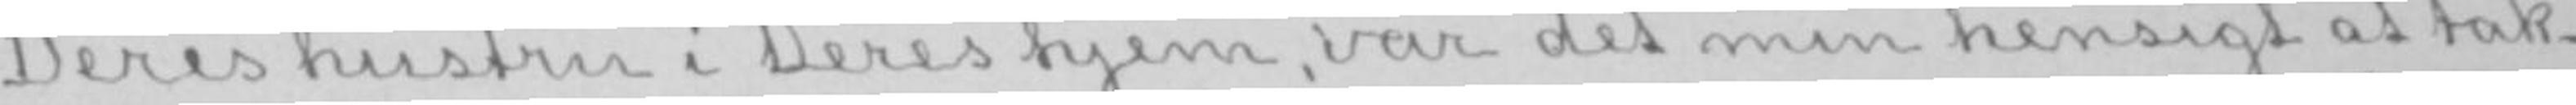Deres hustru i Deres hjem, var det min hensigt at tak-
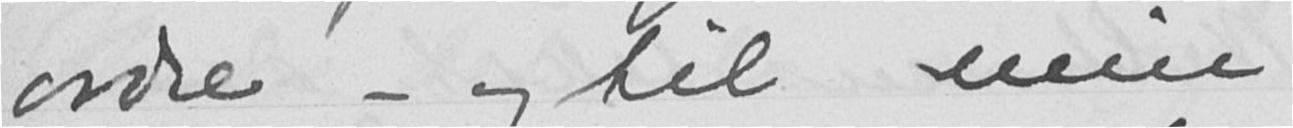ordre - og til min
